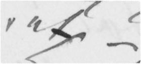et
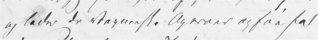og lader de Vagnerske Operaer opføre for
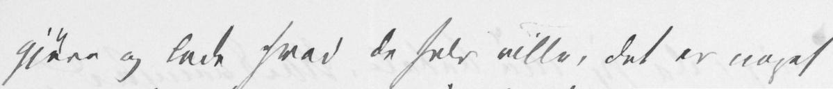gjøre og lade hvad de selv ville, det er noget
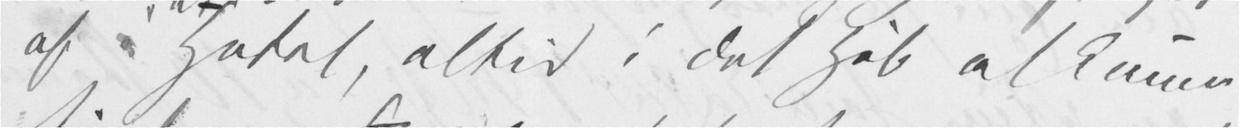af i Hotel, altid i det Håb at kunne
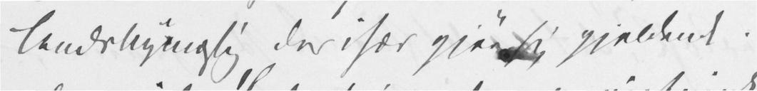Landsbysagtig der især gjør sig gjeldende i
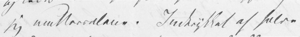sig muttersalene. Indtrykket af halve
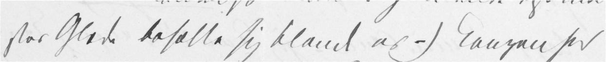stor Glæde bosætte sig blandt os -) Kongen her
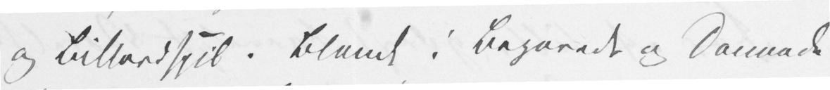og Billardspil. Blandt i Begavede og Dannede
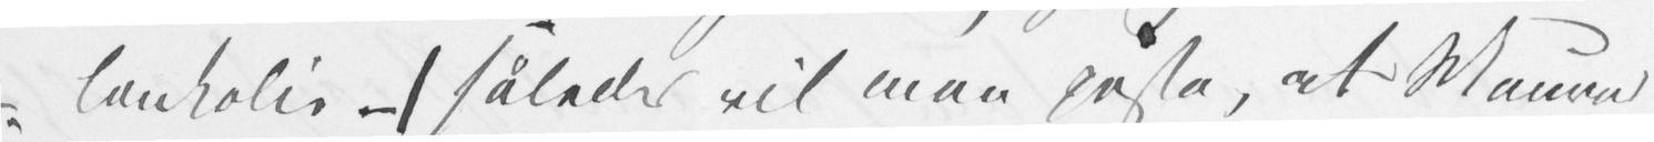lankolie – (således vil man påsta, at Maurer
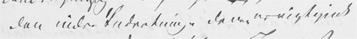den indre Indretning. den er rigtignok
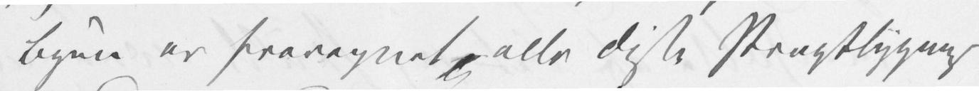Byen er fraregnet, alle disse Pragtbygninger
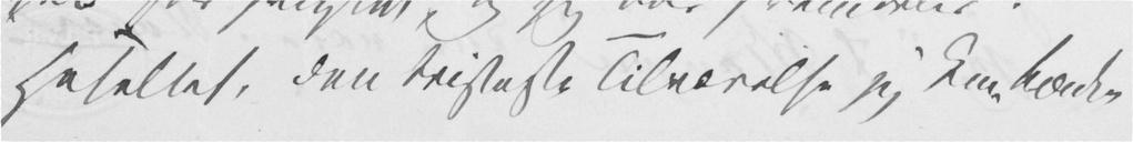Hotellet, den tristeste Tilværelse jeg kan tænke
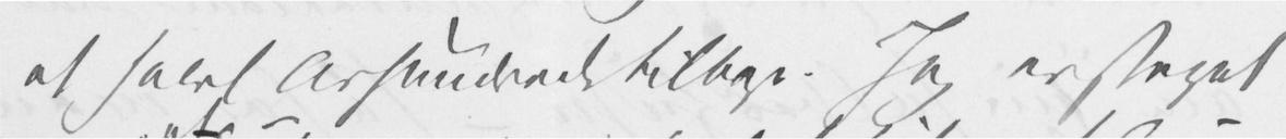et halvt Arhundrede tilbage. Jeg er steget
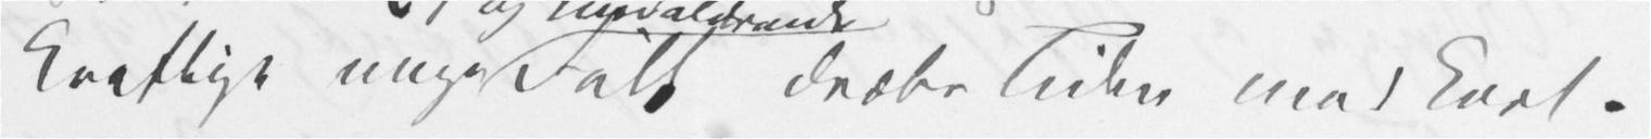kraftige unge Folk dræbe Tiden med Kort-
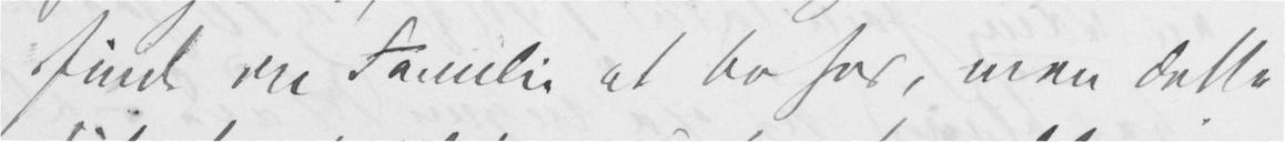finde en Familie at bo hos, men dette
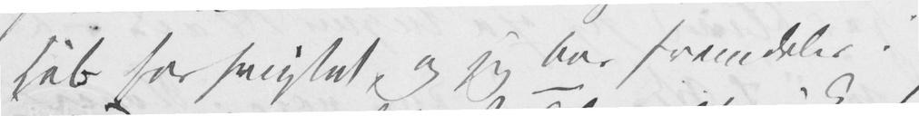Håb har svigtet, og jeg bor fremdeles i
